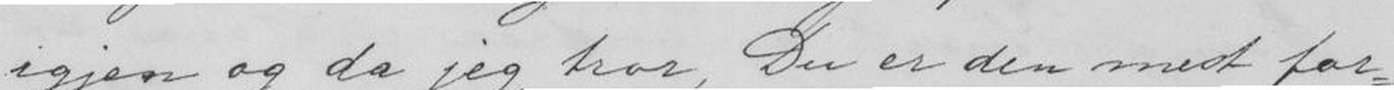igjen og da jeg tror, Du er den mest for-
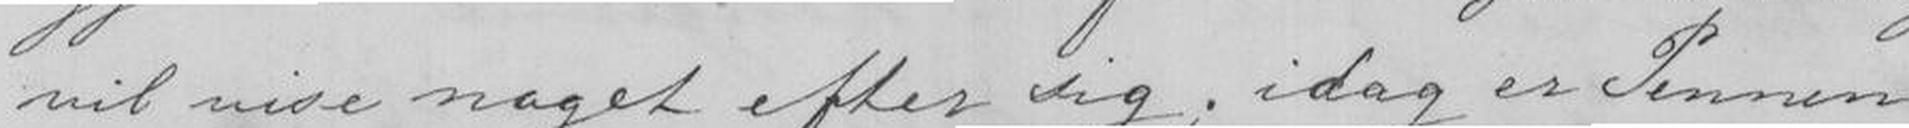vil vise noget efter sig, idag er Pennen
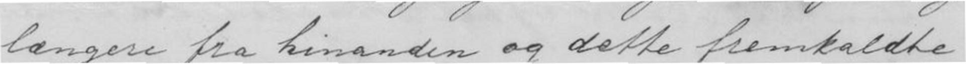længere fra hinanden og dette fremkaldte
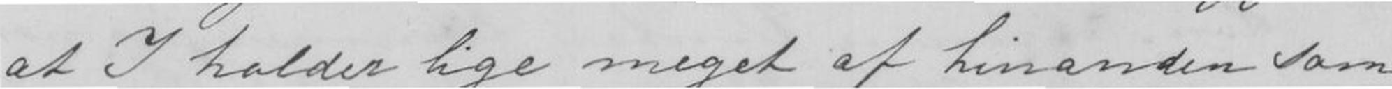at I holder lige meget af hinanden som
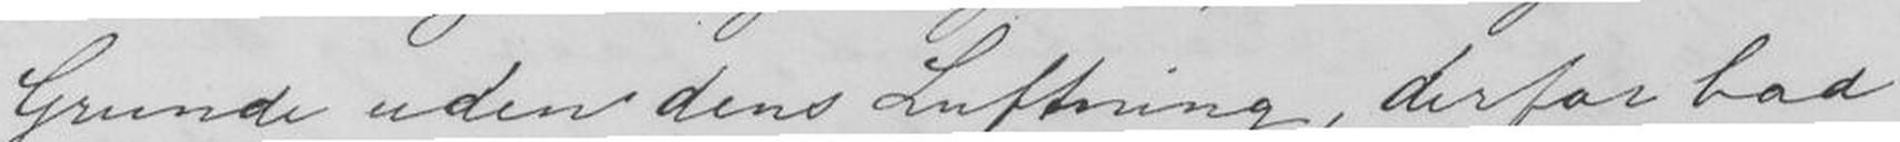Grunde uden dens Luftning, derfor bad
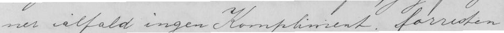ner ialfald ingen Kompliment, forresten
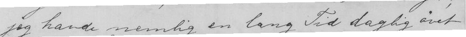jeg havde nemlig en lang Tid daglig øvet
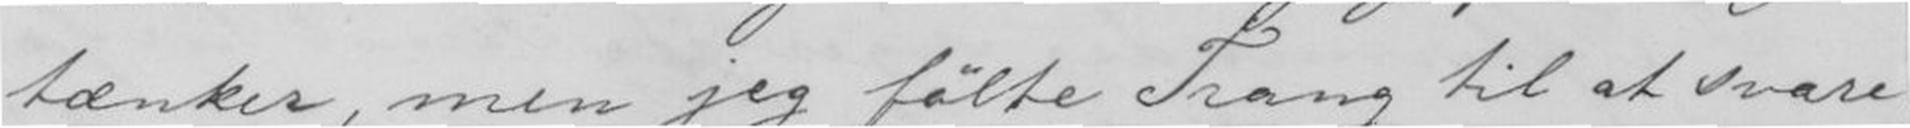tænker, men jeg følte Trang til at svare
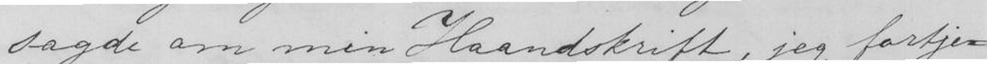sagde om min Haandskrift, jeg fortje-
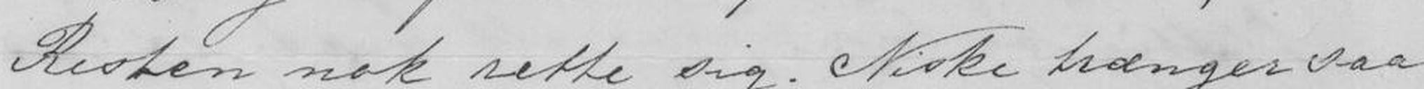Resten nok rette sig. Niske trænger saa
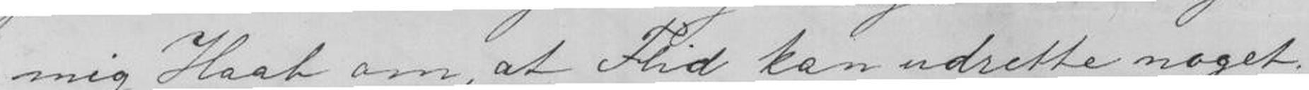mig Haab om, at Flid kan udrette meget;
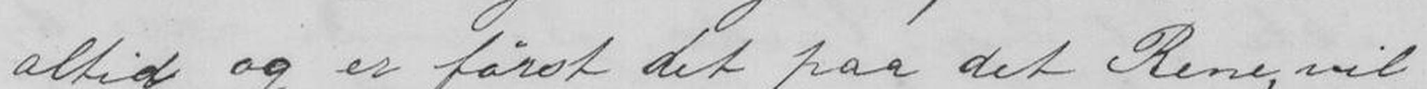altid og er først det paa det Rene, vil
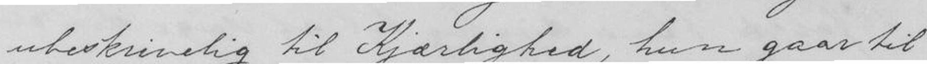ubeskrivelig til Kjærlighed, hun gaar til
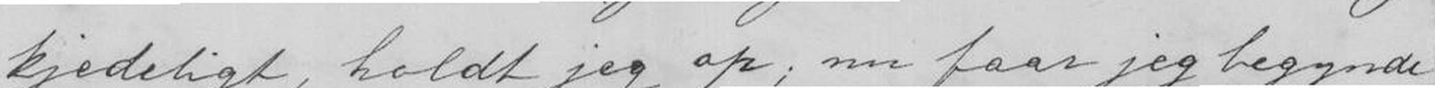kjedeligt, holdt jeg op; nu faar jeg begynde
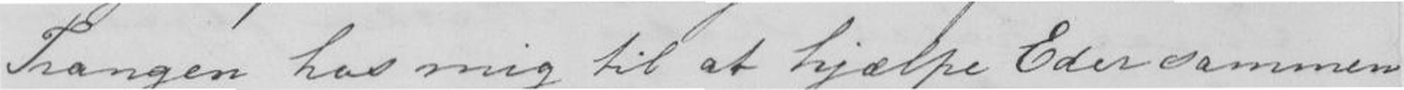Trangen hos mig til at hjælpe Eder sammen
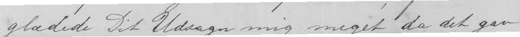glædede Dit Udsagn mig meget da det gav
